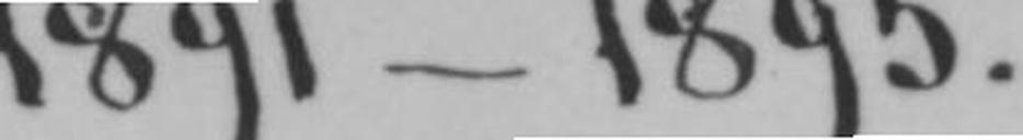1891-1895.
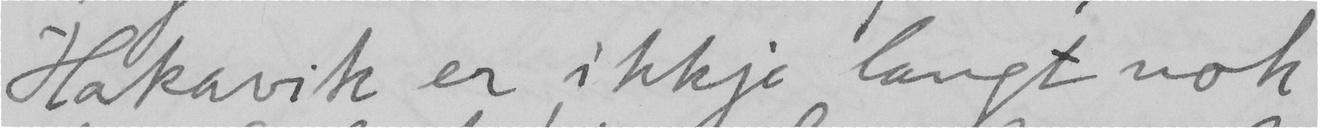Hakavik er ikkje langt nok
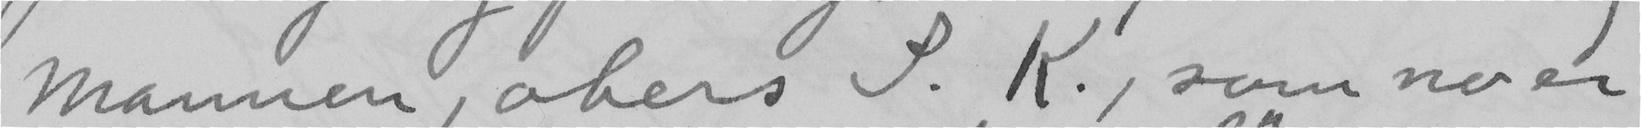mannen, obers S.K., som no er
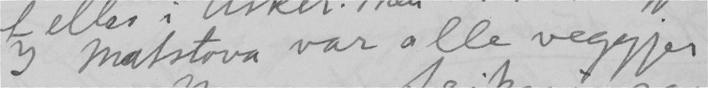I matstova var alle veggjer
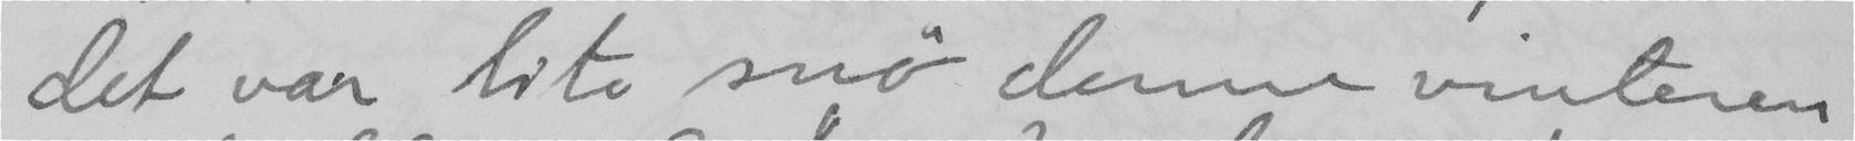det var lite snö denne vinteren
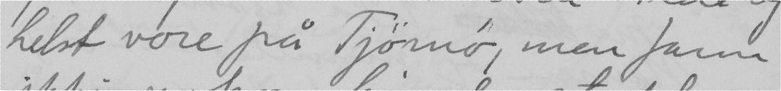helst vore på Tjömö, men fann
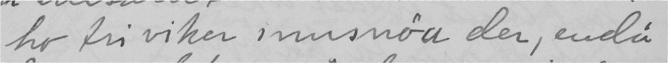ho fri viker innsnöa der, endå
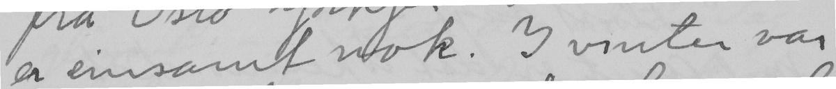er einsamt nok. I vinter var
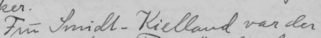Fru Smidt-Kielland var der
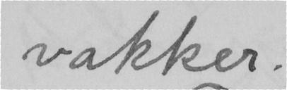vakker.
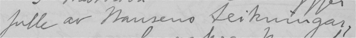fulle av Nansens teikningar,
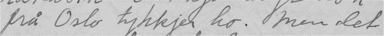frå Oslo tykkjer ho. Men det
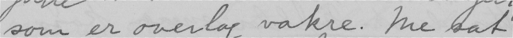som er overlag vakre. Me sat
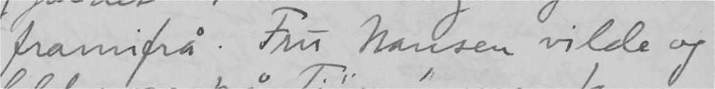framifrå. Fru Nansen vilde og
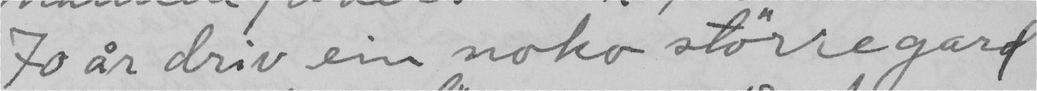70 år driv ein noko större gard
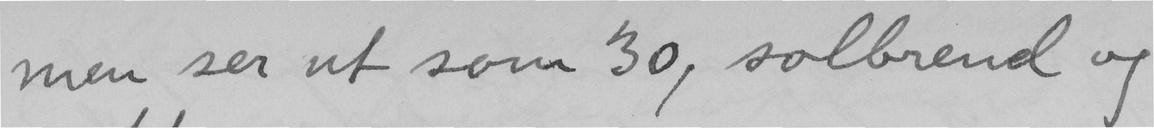men ser ut som 30, solbrend og
